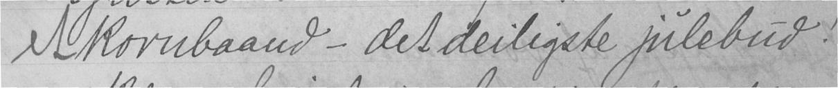et kornbaand - det deiligste julebud!
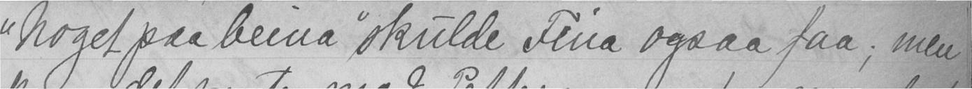"Noget paa beina" skulde Tina ogsaa faa; men
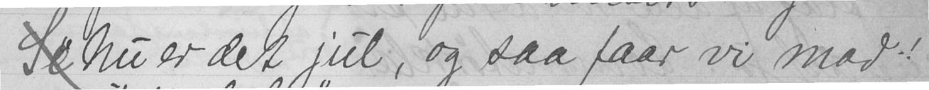Nu er det jul, og saa faar vi mad!
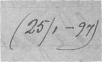(25/1-97)
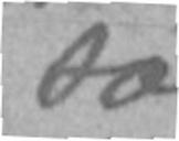saa
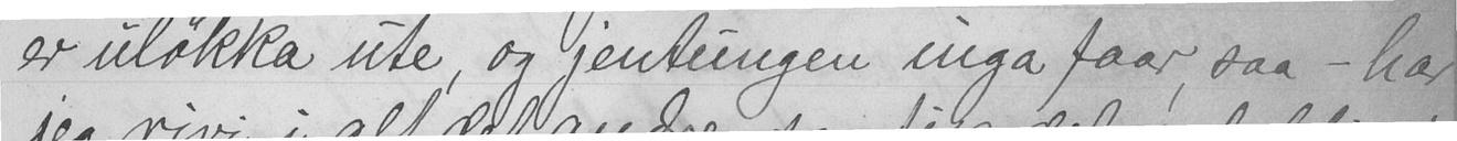er uløkka ute, og jentungen inga faar, saa - har
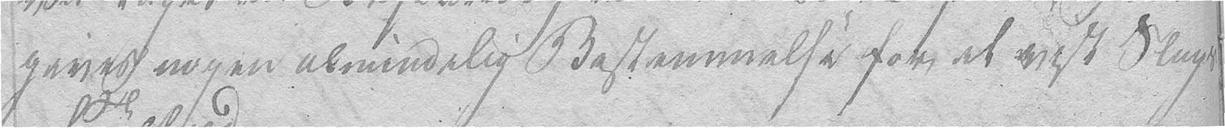gives nogen almindelig Bestemmelse for et vist Slags
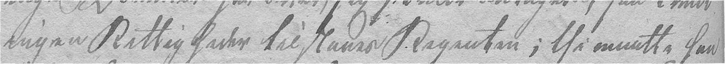ingen Rettigheder tilstaaes Regenten; thi maatte han
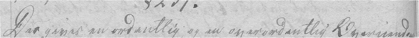Der gives en ordentlig og en overordentlig Overeiendoms-
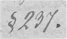§ 237.
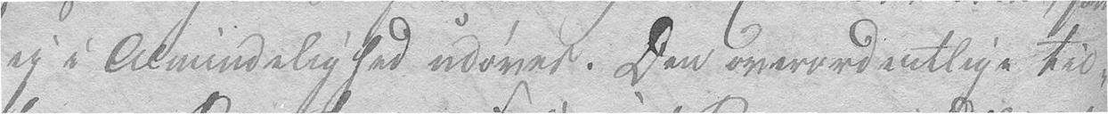ey i Almindelighed udøves. Den overordentlige til-
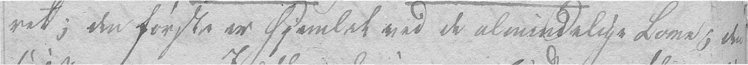ret; den første er hjemlet ved de almindelige Love; den
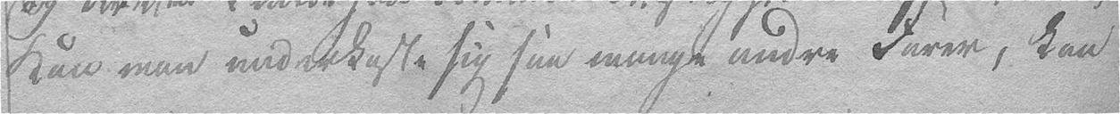Kan man underkaste sig saa mange andre Farer, kan
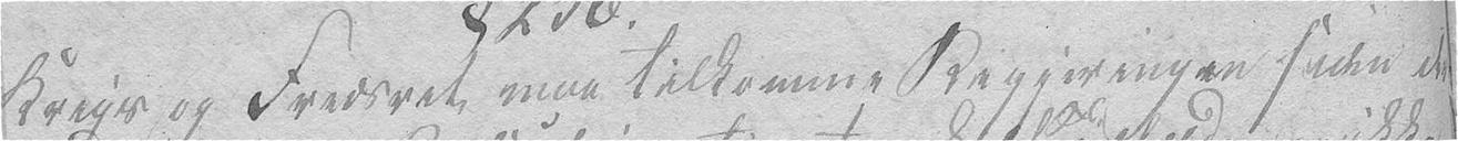Krigs og Fredsret maa tilkommer Regjeringen siden der-
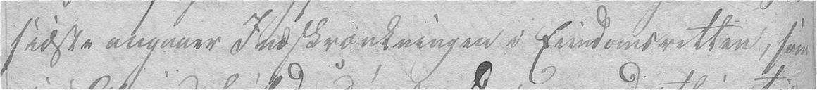sidste angaaer Indskrænkningen i Eiendomsretten, som
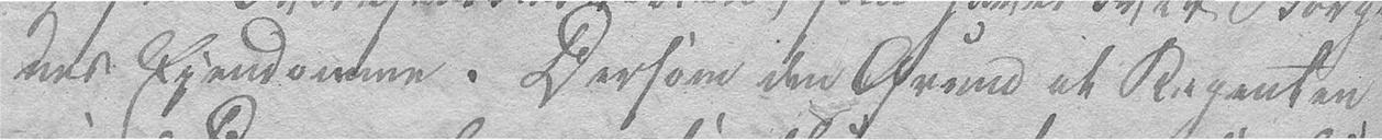nes Eiendomme. Dersom den Grund at Regenten
